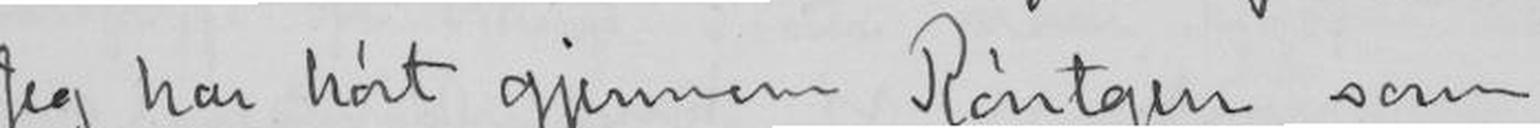Jeg har hørt gjennem Røntgen, som
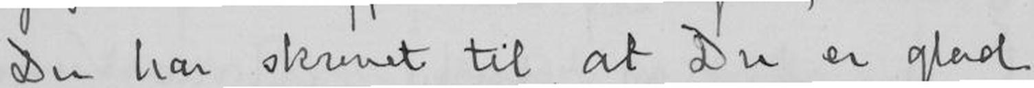Du har skrevet til, at Du er glad
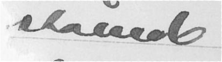stående
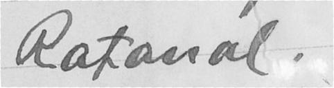Rotanal.
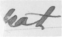lat
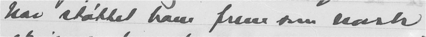har støttet han frem som norsk
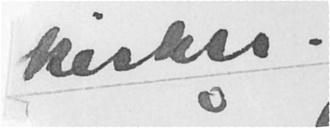kirker.
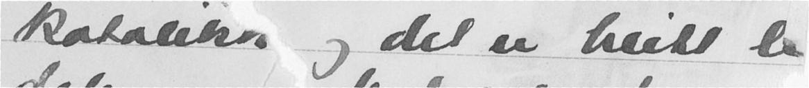katolikk og det er blitt li
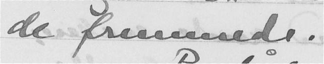de fremmede.
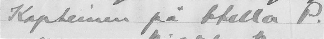Kapteinen pa Stella P.
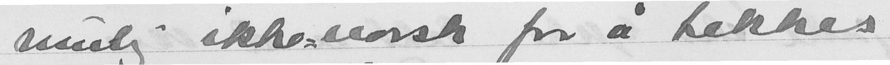mulig ikke-norsk for å tekkes
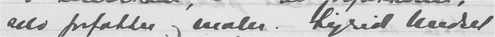selv forfatter og maler. Sigrid Undset
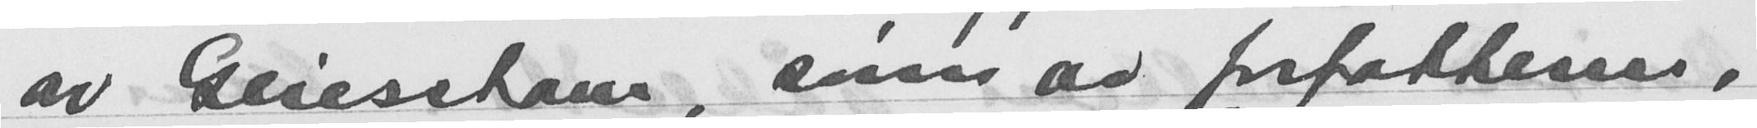av Geierstam, sønn av forfatteren,
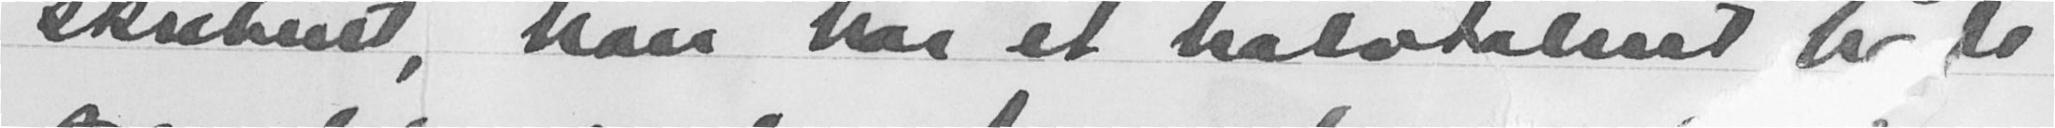skribent, han har et halvtalent både
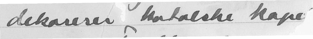dekorerer katolske kape
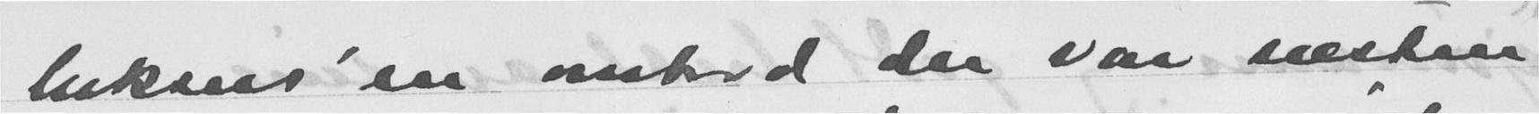luksus'en ombord der var nesten
